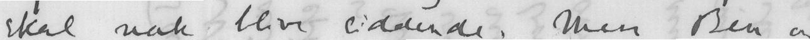skal nok blive siddende. Men Ben og
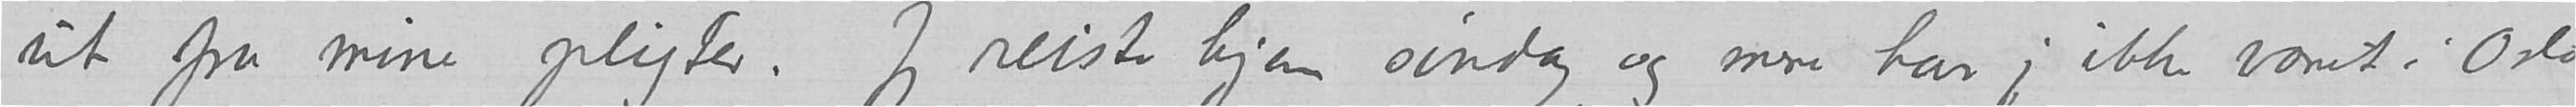ut fra mine pligter. Jeg reiste hjem søndag og mere har jeg ikke været i Oslo
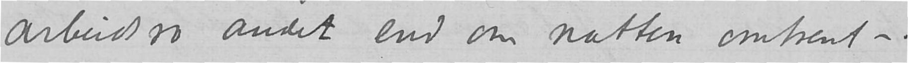arbeidsro andet end om natten omtrent –.
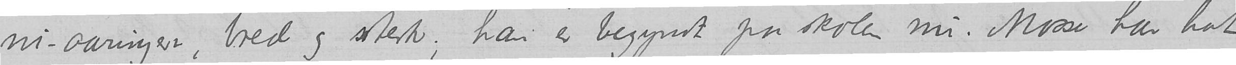ni-aaringer, bred og sterk; han er begyndt paa skolen nu. Mosse har hat
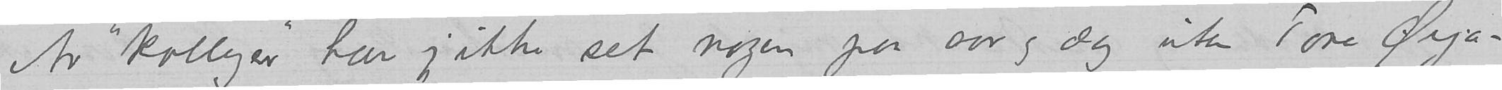Av «kolleger» har jeg ikke set nogen paa aar og dag uten Tore Ørja
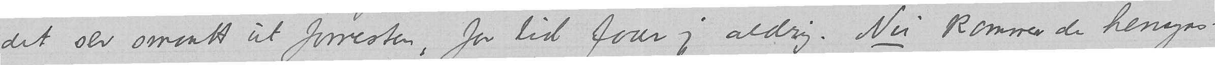det ser smaatt ut forresten, for tid faar jeg aldrig. Nu kommer de hensyns
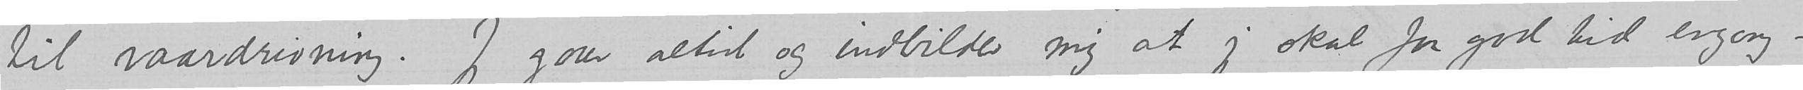til vaardrivning. Jeg gaar altid og indbilder mig at jeg skal faa god tid engang-
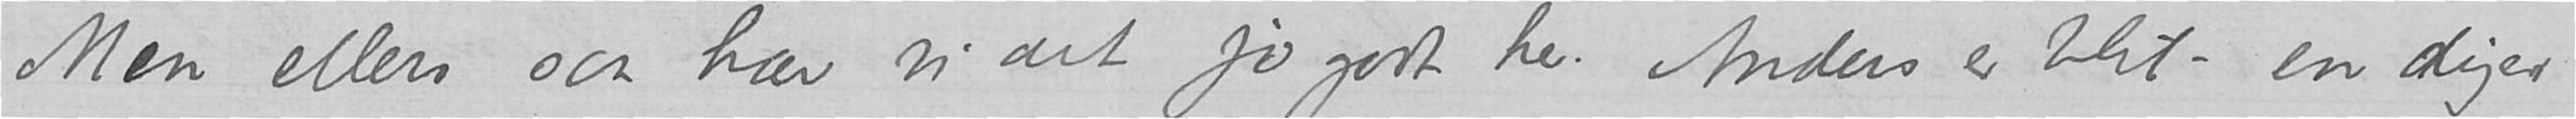Men ellers saa har vi det jo godt her. Anders er blit en diger
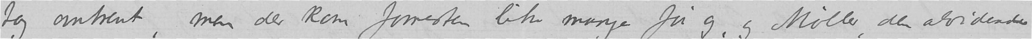tog omtrent, men der kom forresten like mange før og, og Møller, den alvidende
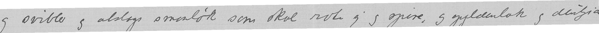og svibler og alslags smaaløk som skal rote sig og spire, og gyldenlak og deutzia
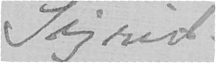Sigrid
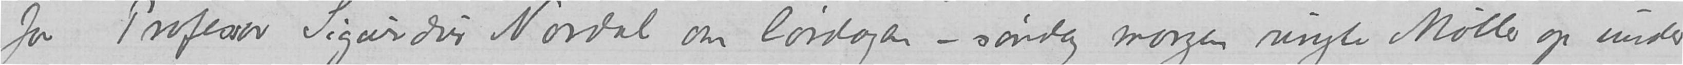for Professor Sigurdur Nordal om lørdagen – søndag morgen ringte Møller op
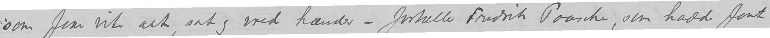som faar vite alt, sat og vred hænder – fortæller Fredrik Paasche, som hadde faat
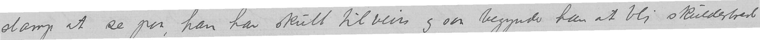slamp at se paa, han har skutt tilveirs og saa begynder han at bli skulderbred
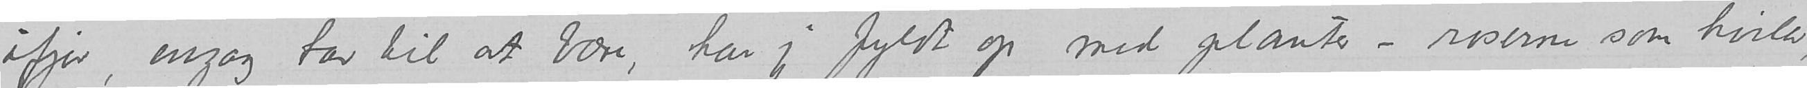ifjor, engang tar til at bære, har jeg fyldt op med planter – roserne som hviler,
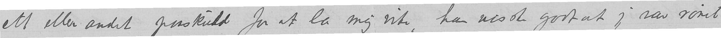under ett eller andet paaskudd for at la mig vite, han visste godt at jeg var røret
